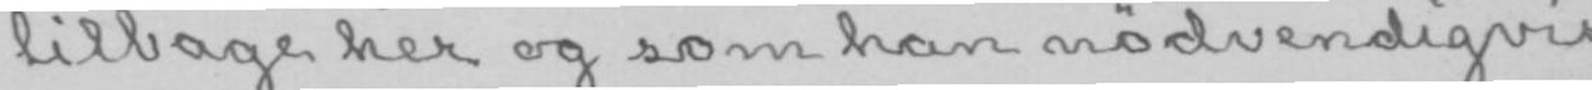tilbage her og som han nødvendigvis
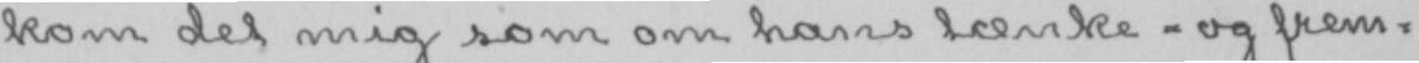kom det mig som om hans tænke- og frem-
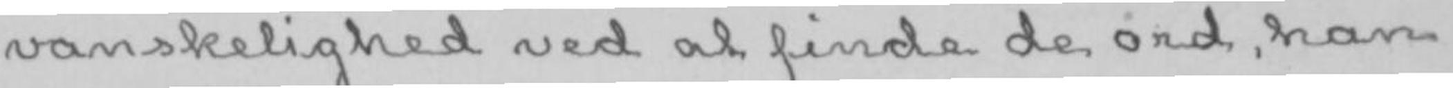vanskelighed ved at finde de ord, han
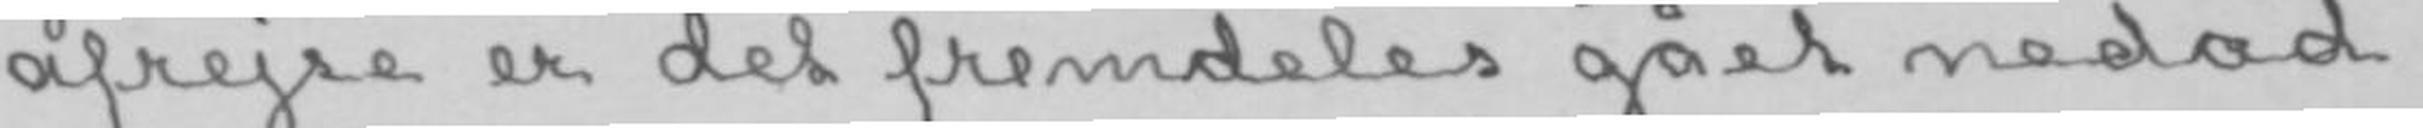afrejse er det fremdeles gået nedad
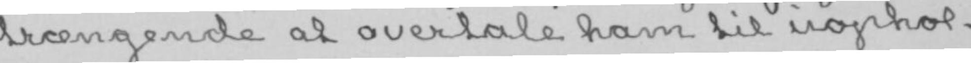trængende at overtale ham til uophol-
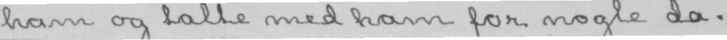ham og talte med ham for nogle da-
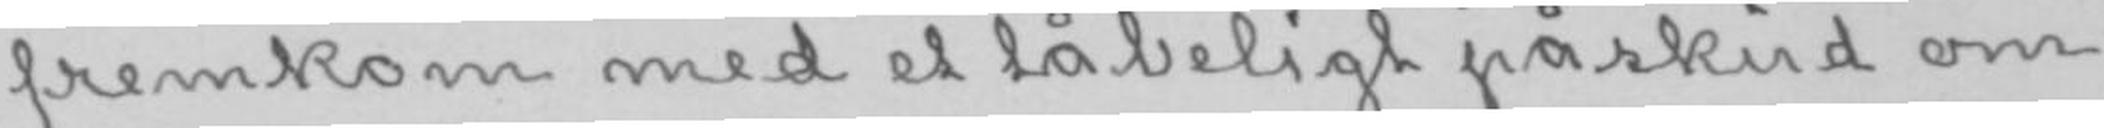fremkom med et tåbeligt påskud om
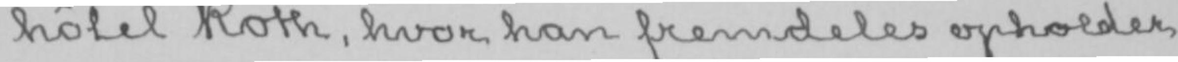hôtel Roth, hvor han fremdeles opholder
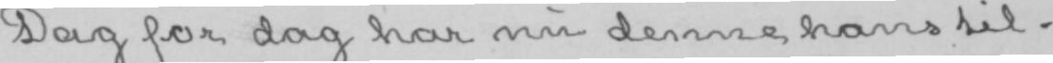Dag for dag har nu denne hans til-
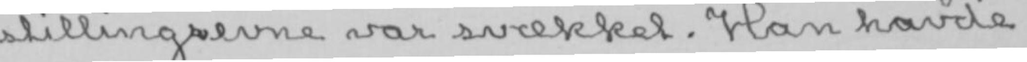stillingsevne var svækket. Han havde
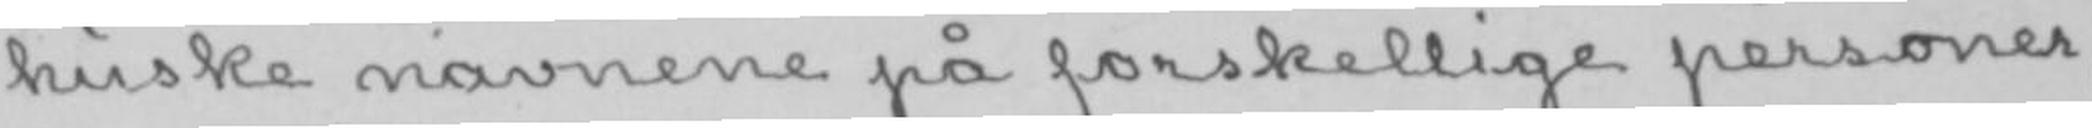huske navnene på forskellige personer,
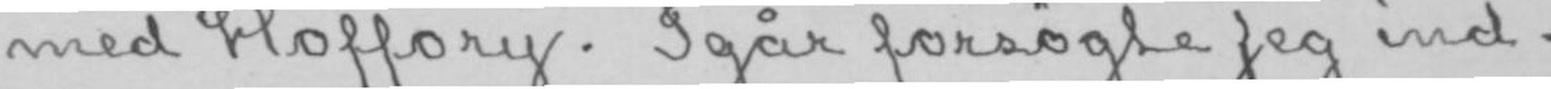med Hoffory. Igår forsøgte jeg ind-
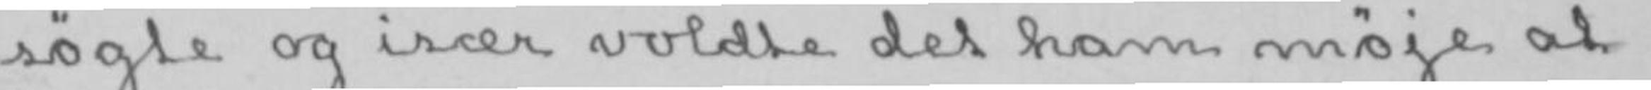søgte og især voldte det ham møje at
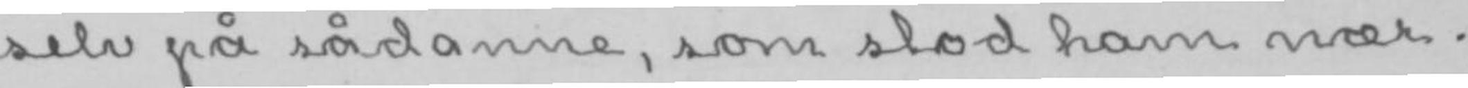selv på sådanne, som stod ham nær.
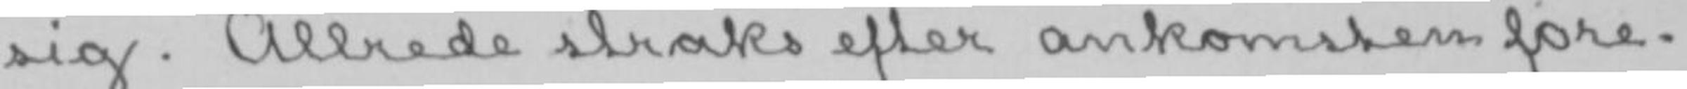sig. Allrede straks efter ankomsten fore-
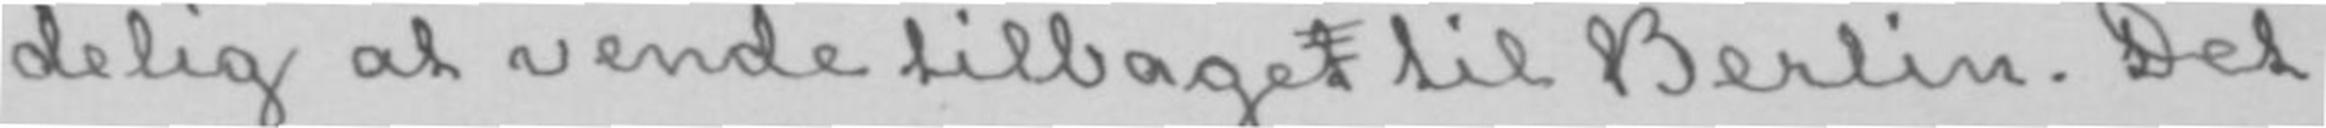delig at vende tilbaget til Berlin. Det
